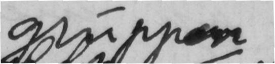gruppen
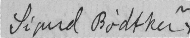Sigud Bødtker
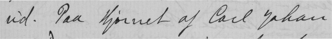ud. Paa Hjørnet af Carl Johan
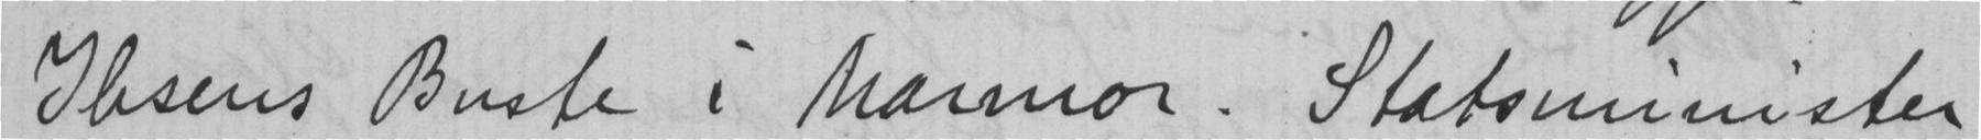Ibsens Buste i Marmor. Statsminister
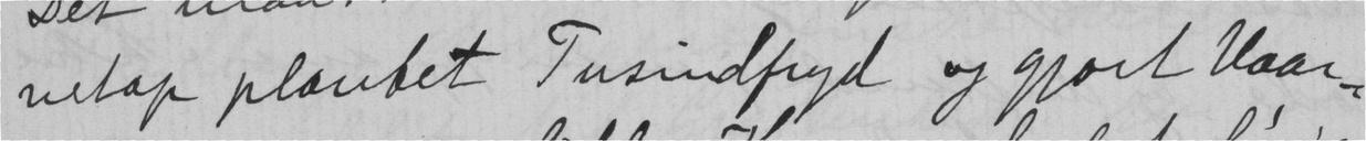netop plantet Tusindfryd og gjort Vaar-
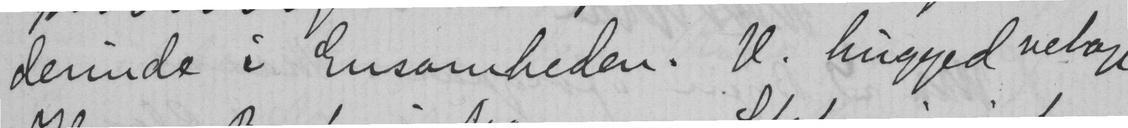derinde i Ensomheden. V. hugged nelop
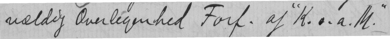vældig Overlegenhed Forf. af "K. s. a. M."
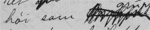høi som
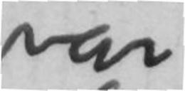var
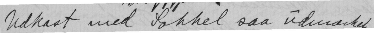Udkast med Sokkel saa udmærket
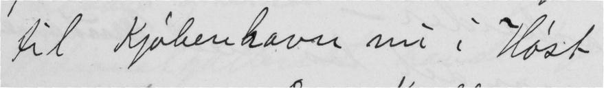til Kjøbenhavn nu i Høst
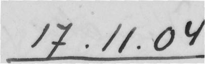17.11.04
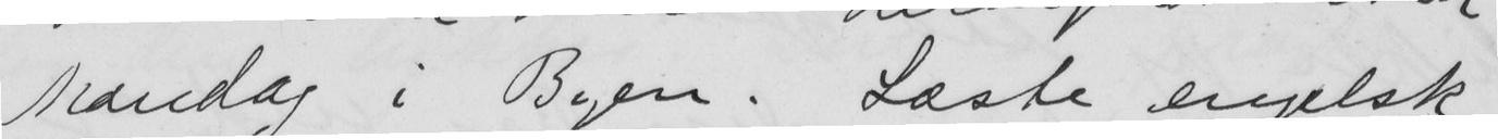Mandag i Byen. Læste engelsk
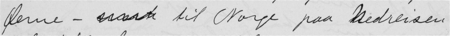Øerne -  til Norge paa Nedreisen
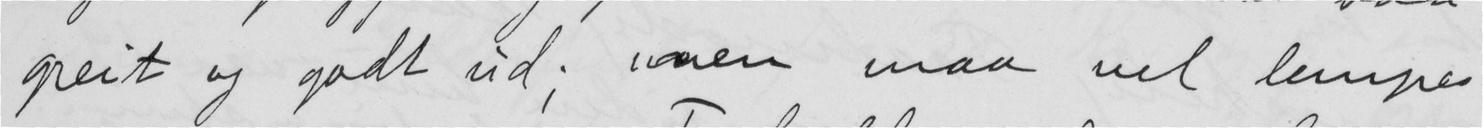greit og godt ud; men maa vel lempe
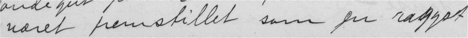været fremstillet som en ragget
